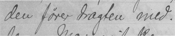den fører dragten med.
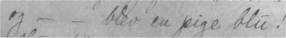og - - blev en pige blu!
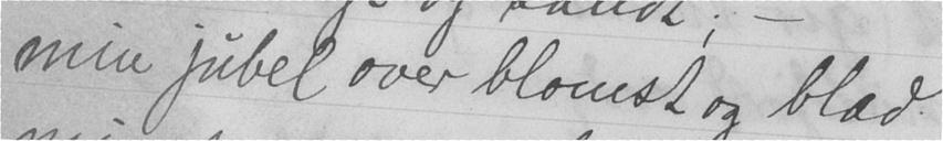min jubel over blomst og blad
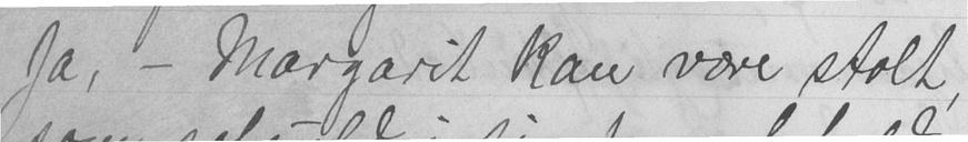Ja, - Margarit kan være stolt,
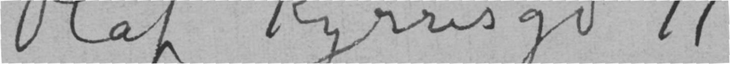Olaf Kyrresgd 11
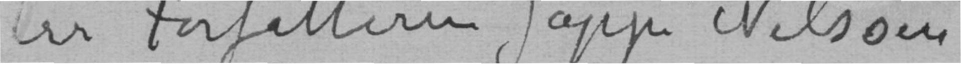Hrr Forfatteren Jappe Nilssen
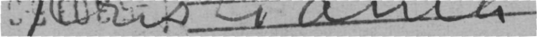Kristiania
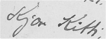Kjære Kitti,
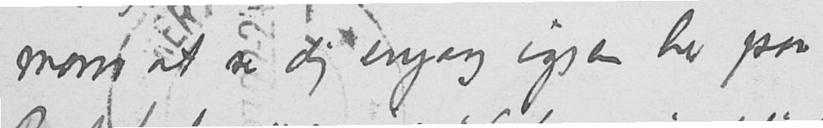morro at se dig engang igjen her paa
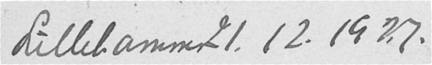Lillehammer 1.12.1927.
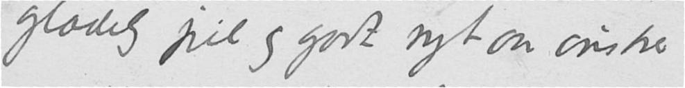glædelig jul og godt nytaar ønsker
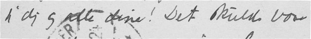jeg dig og alle dine! Det skulde være
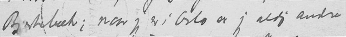Bjerkebæk; naar jeg er i Oslo ser jeg aldrig andre
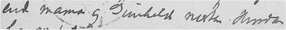end mama og Gunhilds næsten. Hvordan
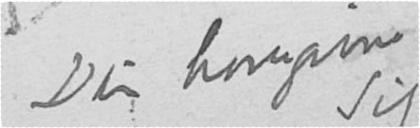Din hengivne
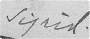Sigrid.
